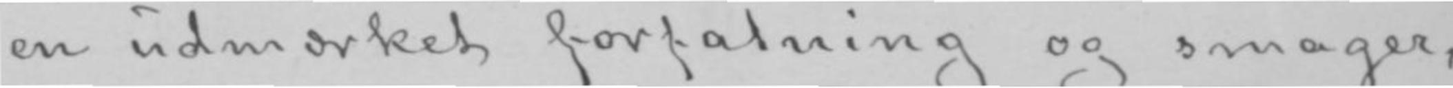en udmærket forfatning og smager,
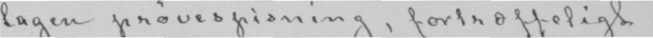tagen prøvespisning, fortræffeligt.
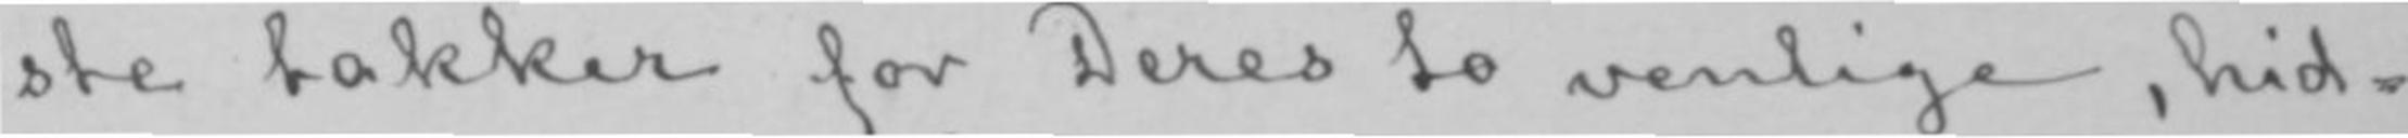ste takker for Deres to venlige, hid-
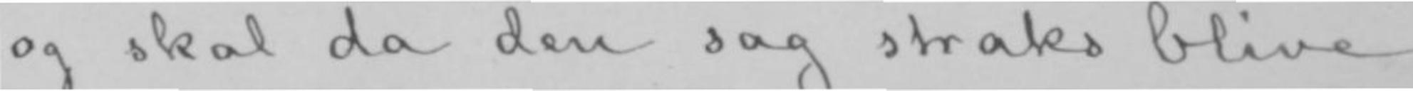og skal da den sag straks blive
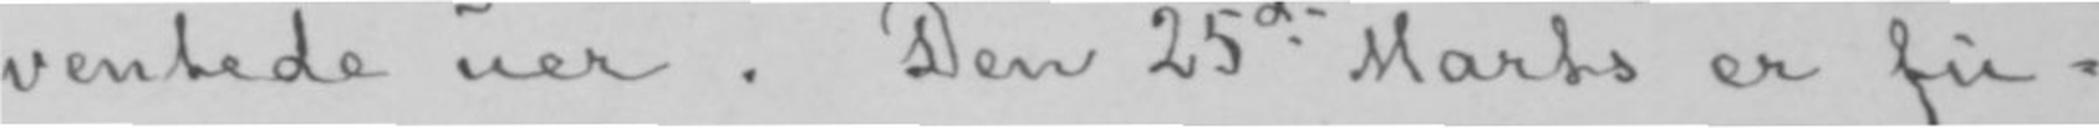ventede uer. Den 25de Marts er fu-
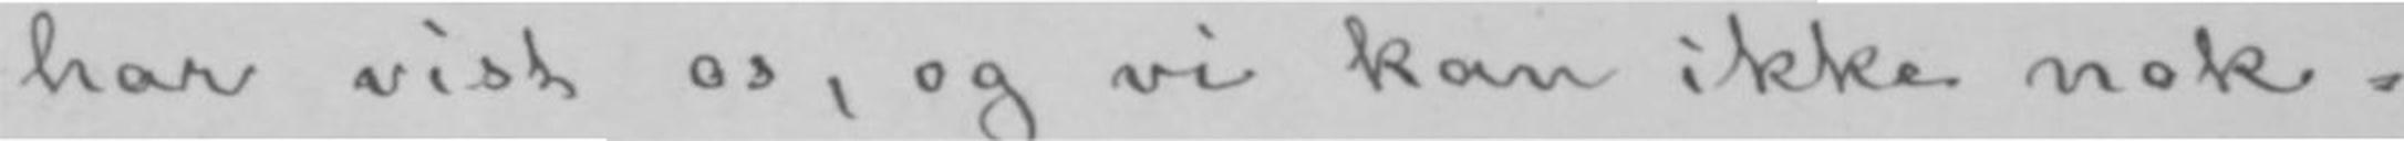har vist os, og vi kan ikke nok-
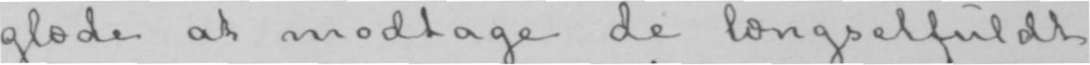glæde at modtage de længselfuldt
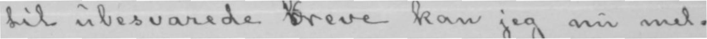til ubesvarede breve kan jeg nu mel-
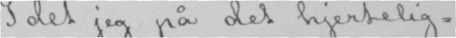Idet jeg på det hjertelig-
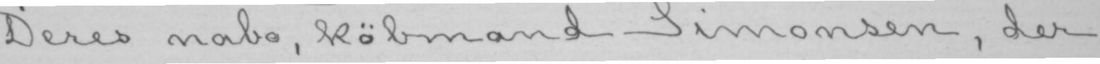Deres nabo, købmand Simonsen, der
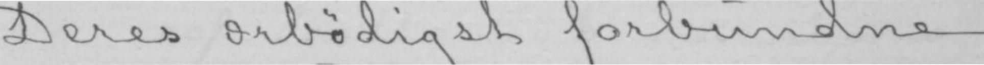Deres ærbødigst forbundne
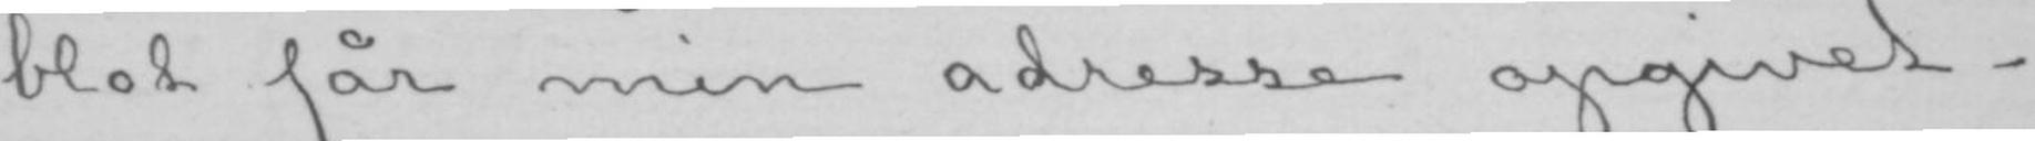blot får min adresse opgivet.
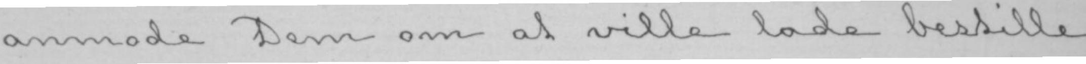anmode Dem om at ville lade bestille
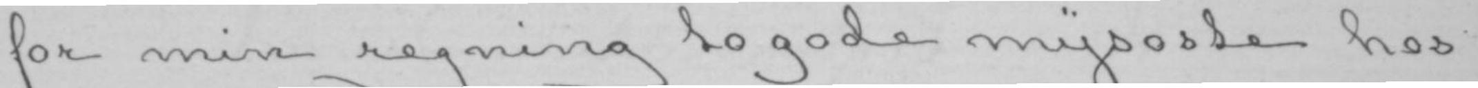for min regning to gode mysoste hos
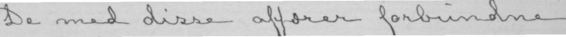De med disse affærer forbundne
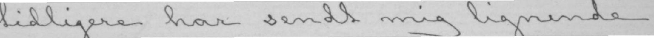tidligere har sendt mig lignende,
